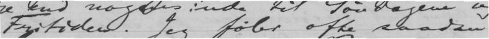Fritiden. Jeg føler ofte saadan
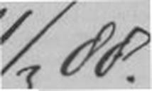11/3 88.
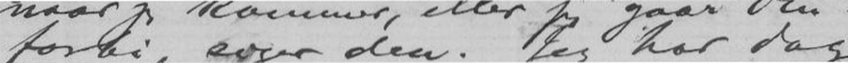forbi, siger den. Jeg har dog
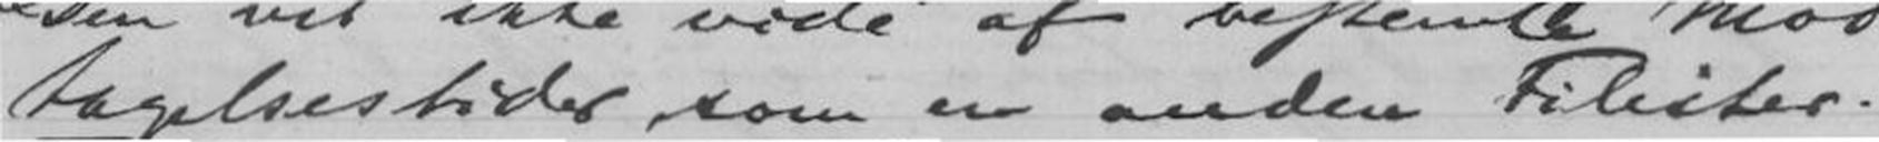tagelsestider som en anden Filister.
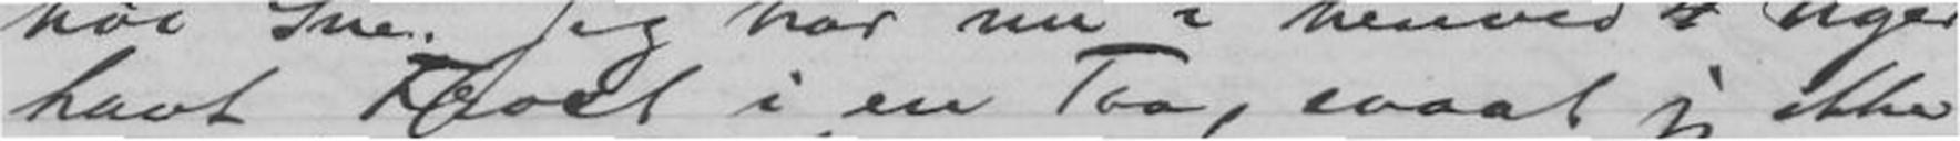havt Frost i en Taa, saaat jeg ikke
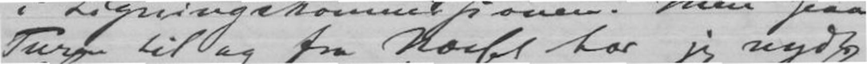Turen til og fra Næsset har jeg nydt
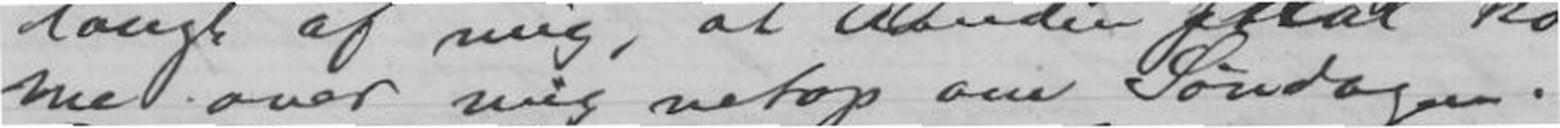me over mig netop om Søndagen.
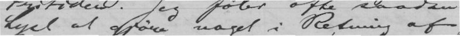Lyst at gjøre noget i Retning af
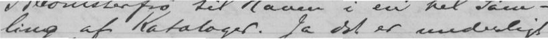ling af Kataloger. Ja det er underligt
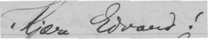Kjære Edvard!
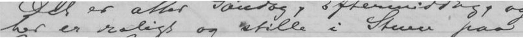her er roligt og stille i Stuen paa
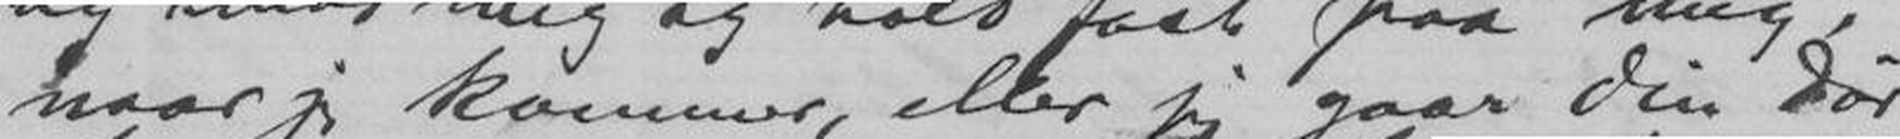naar jeg kommer, eller jeg gaar Din Dør
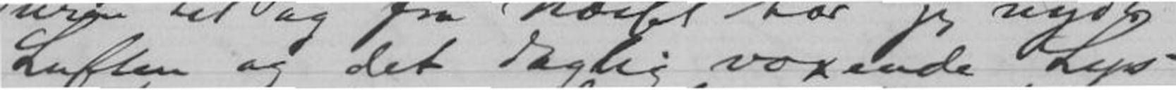Luften og det daglig voxende Lys
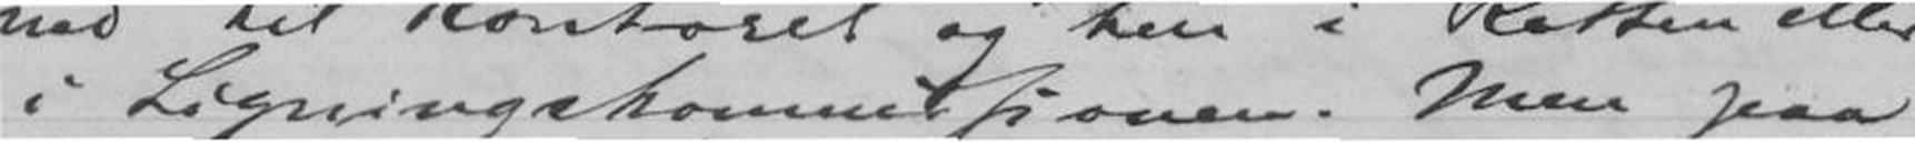i Ligningskommissionen. Men paa
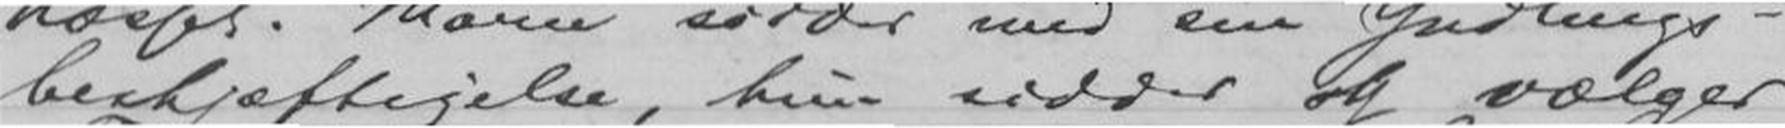beskjeftigelse, hun sidder og vælger
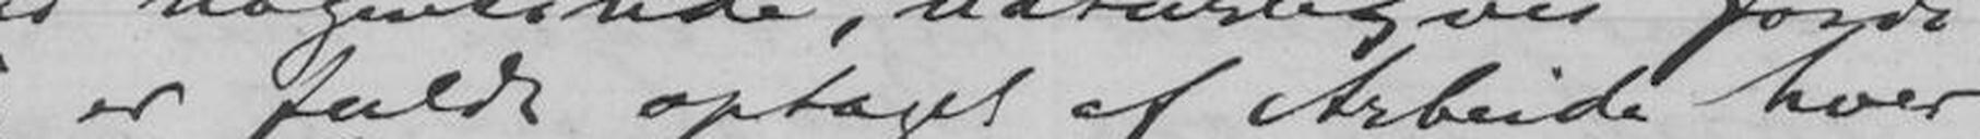jeg er fuldt optaget af Arbeide hver
